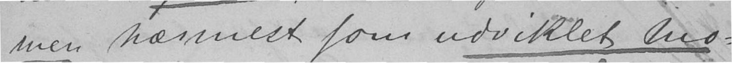men nærmest som udviklet mo-
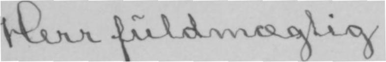Herr fuldmægtig
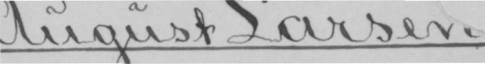August Larsen
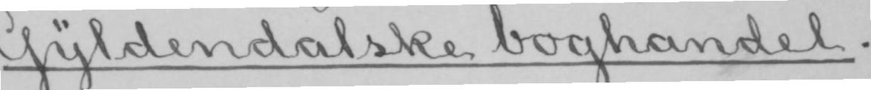Gyldendalske boghandel.
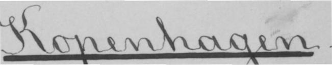Kopenhagen.
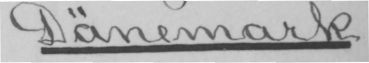Dänemark.
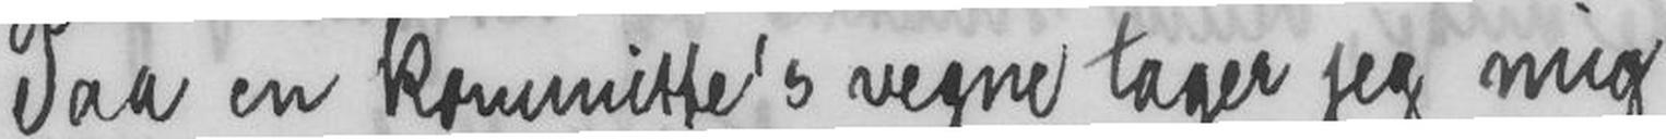Paa en kommitte's vegne tager jeg mig
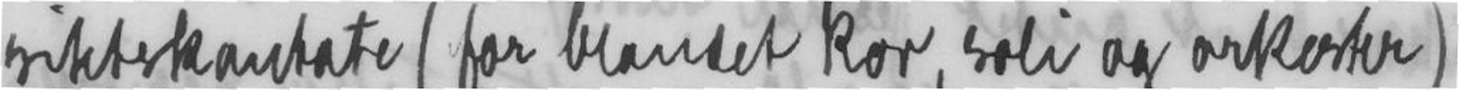sitetskantate (for blandet kor, soli og orkester)
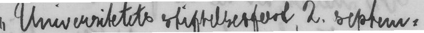"Universitetets stiftelsesfest, 2 septem-
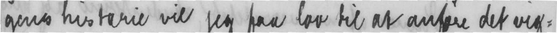gens historie vil jeg faa lov til at anføre det vig-
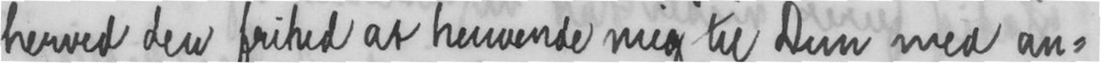herved den frihed at henvende mig til Den med an-
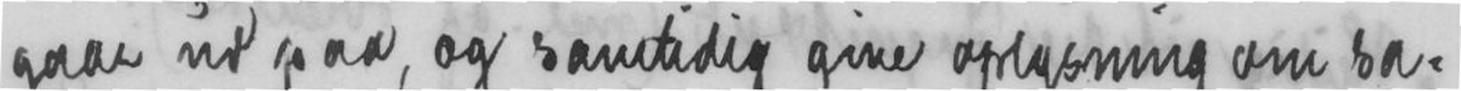gaar ud paa, og samtidig give oplysning om sa-
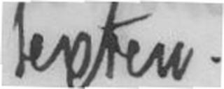tegsten.
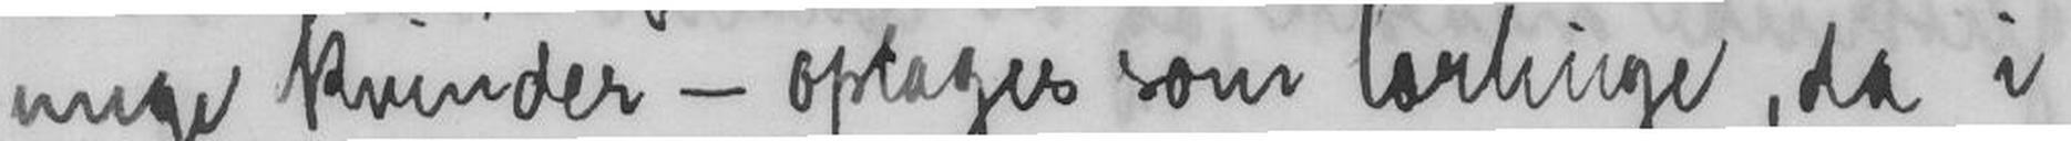unge kvinder - optages som lærlinge, da i
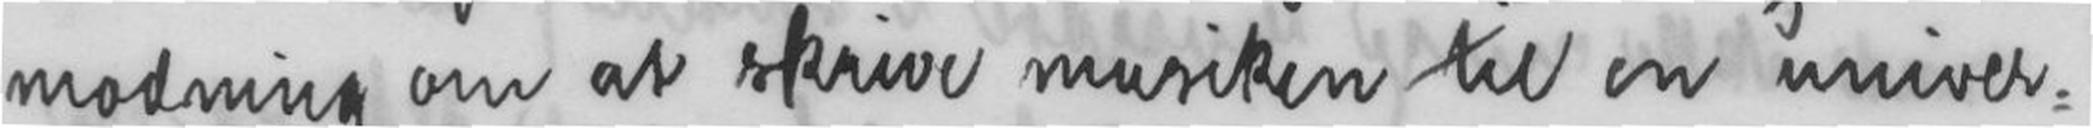modning om at skrive musiken til en univer-
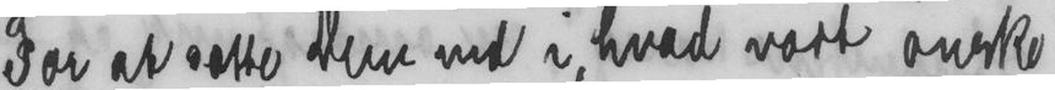For at sætte Dem ind i, hvad vort ønske
